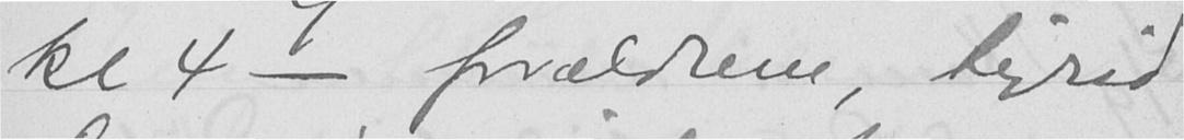kl 4 - forældrene, Sigrid
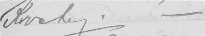Krosby. -
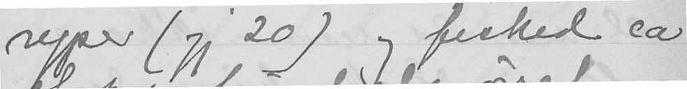ryper (jeg 20) og fisked ca
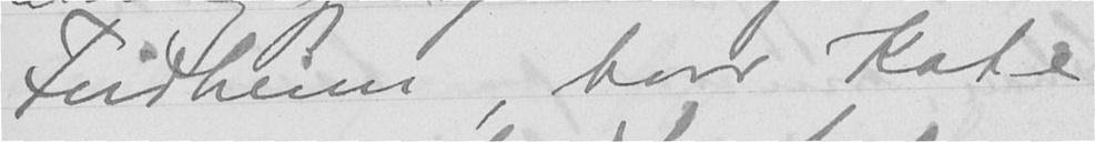Fridheim, hvor Kate
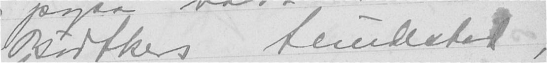Bødtkers, Lundestad,
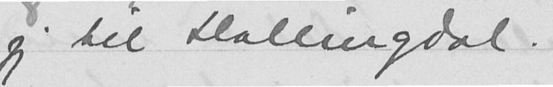jeg til Hallingdal.
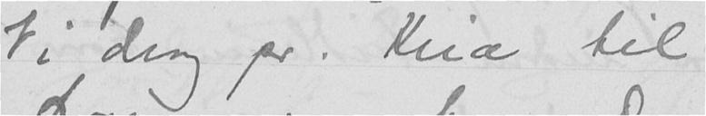Vi drog pr. Kria til
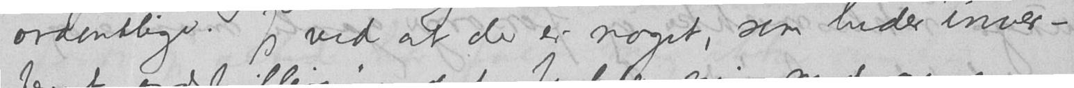ordentlige. Jeg ved at der er noget, som heder inver-
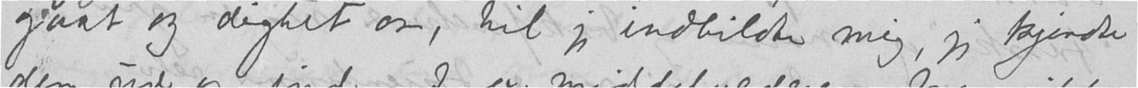gaat og digtet om, til jeg indbildte mig, jeg kjendte
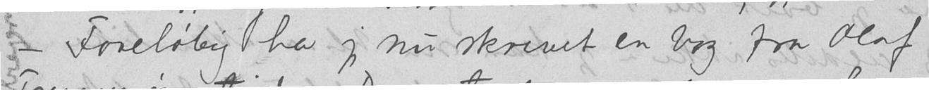- Foreløbig har jeg nu skrevet en bog fra Olaf
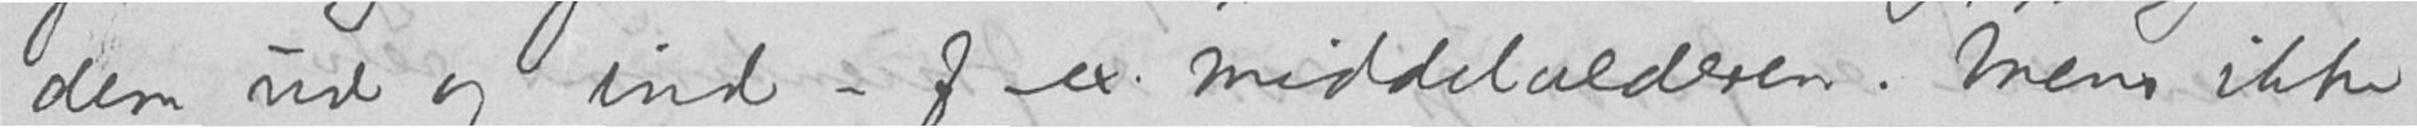dem ud og ind – f. ex. middelalderen. Mens ikke
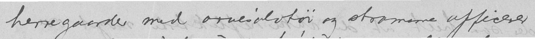herregaarde med arvesølvtøi og stramme officerer
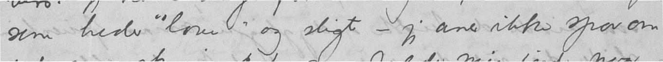som heder "love" og sligt - jeg aner ikke spor om
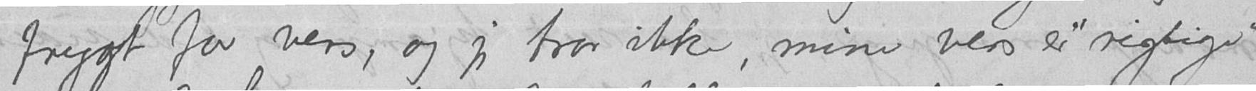frygt for vers, og jeg tror ikke, mine vers er "rigtige"
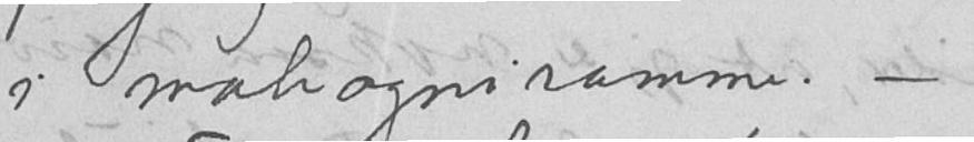i mahogniramme. -
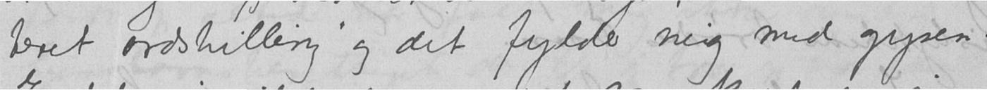teret ordstilling og det fylder mig med gysen.
